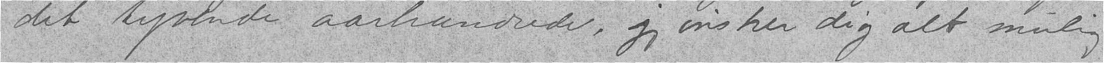det tyvende aarhundrede, jeg ønsker dig alt mulig
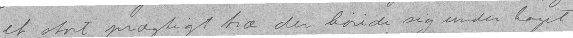et stort prægtigt træ der bøide sig under taget,
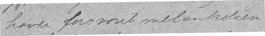havde forsvoret melankolien!
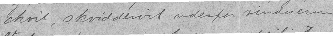skvit, skviddervit udenfor vinduerne.
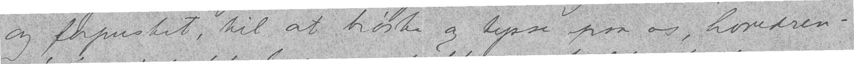og forpustet, til at trøste og hysse paa os, hovedren-
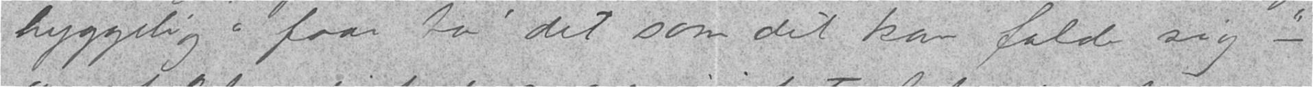hyggelig "faar ta' det som det kan falde sig —"
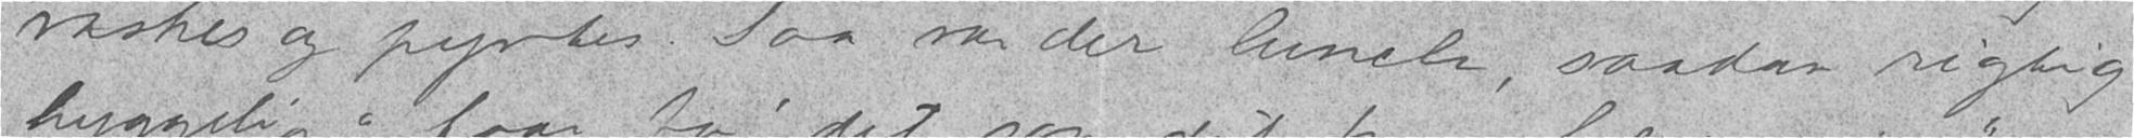vaskes og pyntes. Saa var der lunch, saadan rigtig
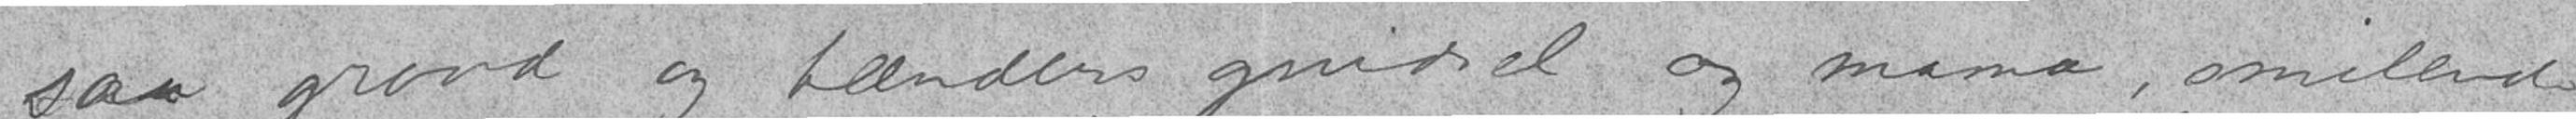saa graad og tænders gnidsel og mama, smilende
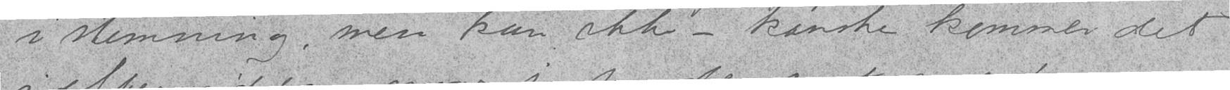i stemning, men kan ikke — kanske kommer det
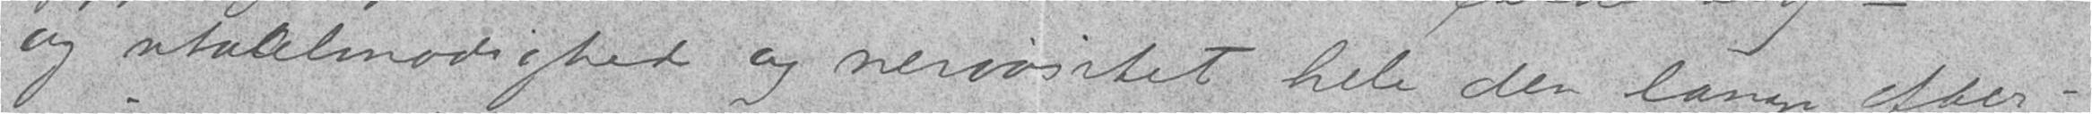og utaalmodighed og nervøsitet hele den lange efter-
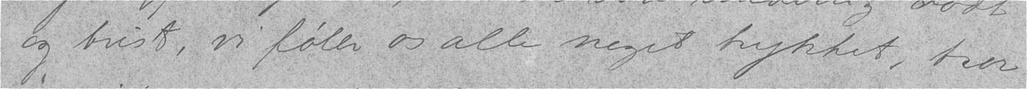og trist, vi føler os alle meget trykket, tror
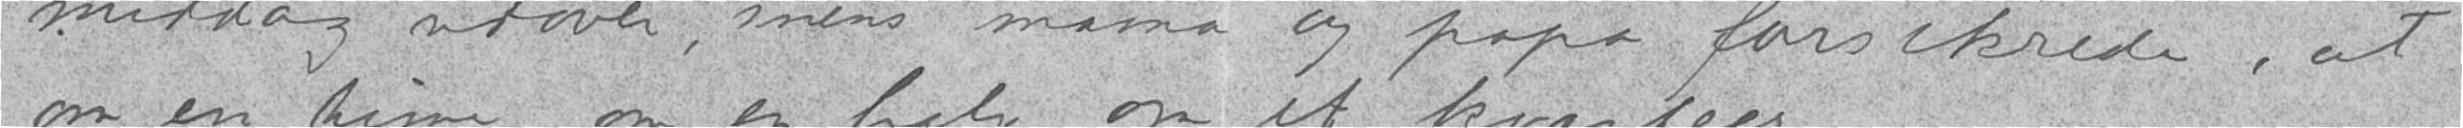middag udover, mens mama og papa forsikrede, at
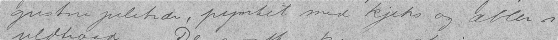gustne juletrær, pyntet med kjeks og æbler i
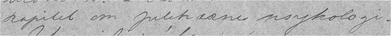kapitel om juletrærnes psykologi —
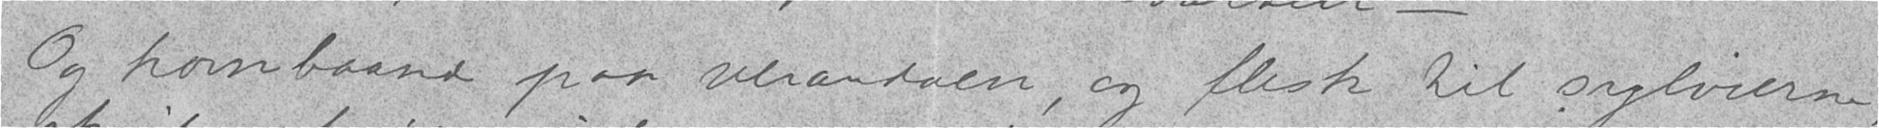Og kornbaand paa verandaen, og flesk til sylvierne,
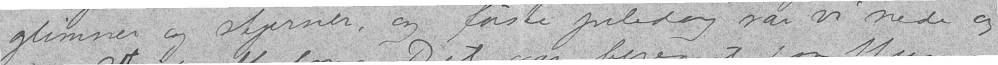glimmer og stjerner, og første juledag var vi nede og
